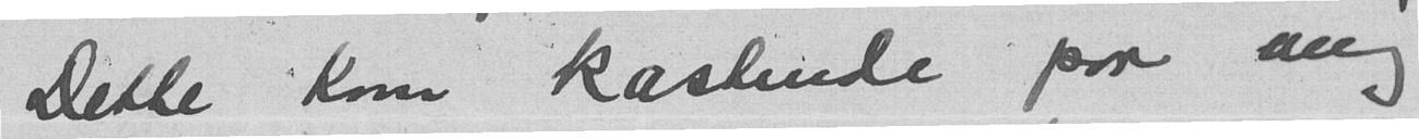Dette kom kastende paa mig
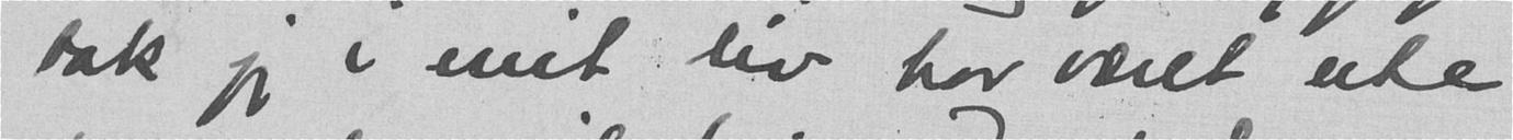bok jeg i mit liv har været ute
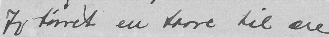Jeg tørret en taare til ære
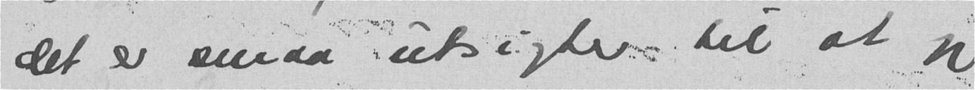det er smaa utsigter til at jeg
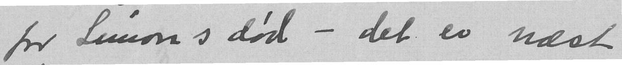for Simons død - det er næst
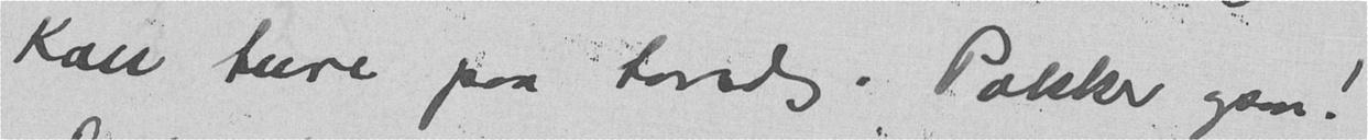kan ture paa torsdag. Pokker ogsaa!
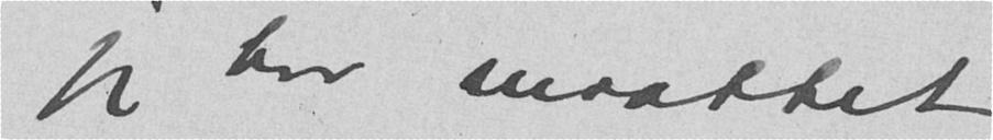jeg har maattet
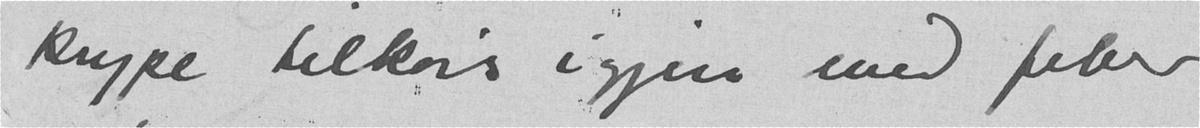krype tilkors igjen med feber
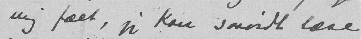mig fælt, jeg kan saavidt læse
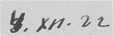4. XII. 22
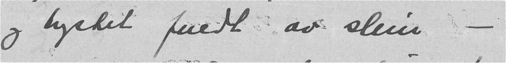og brystet fuldt av slim -
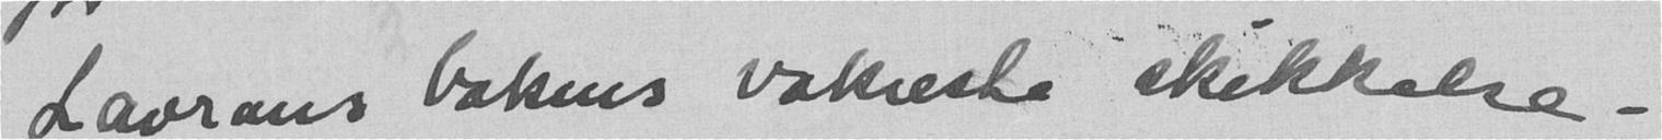Lavrans bokens vakreste skikkelse.
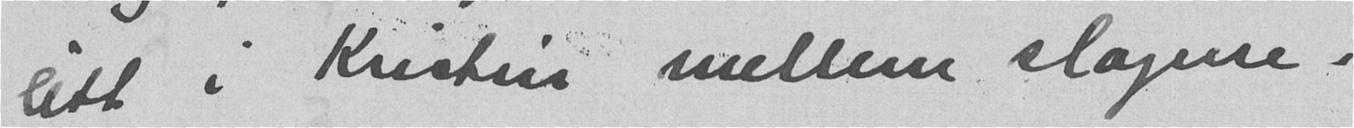litt i Kristin mellem slagene.
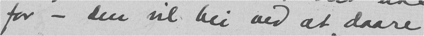for - den vil bli ved at daare
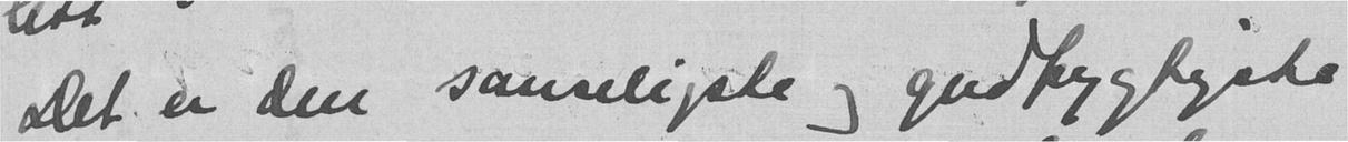Det er den sanseligste og gudfrygtigste
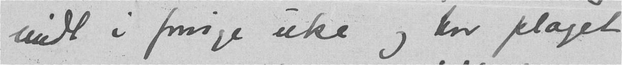midt i forrige uke og har plaget
